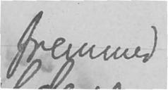fremmed
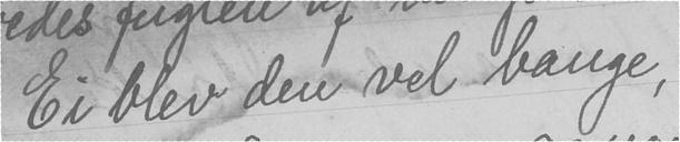Ei blev den vel bange,
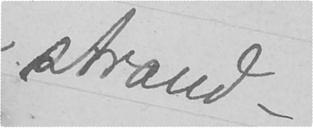strand -
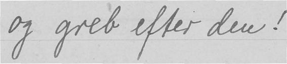og greb efter den!
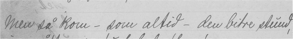Men så kom - som altid - den bitre stund,
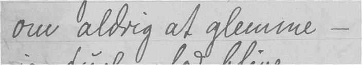om aldrig at glemme -
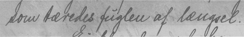som tæredes fuglen af længsel.
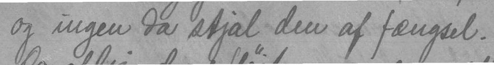og ingen da stjal den af fængsel.
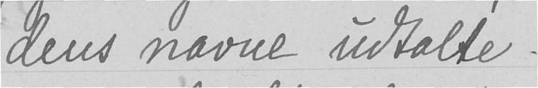dens navne udtalte
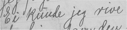Ei kunde jeg rive
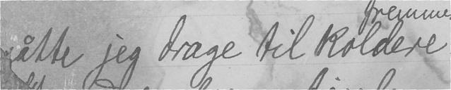måtte jeg drage til koldere
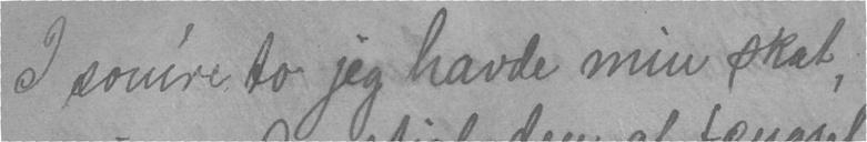I som're to jeg havde min skat,
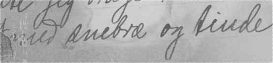med snebræ og tinde
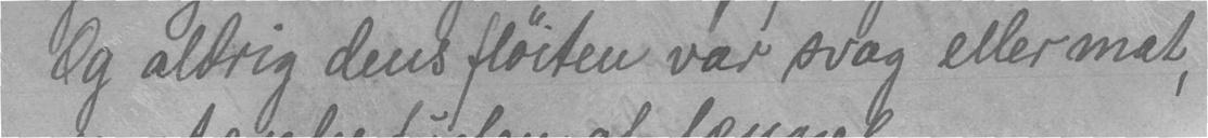Og aldrig dens fløiten var svag eller mat,
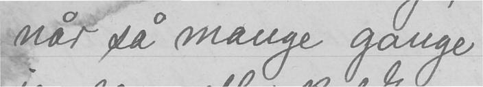når så mange gange
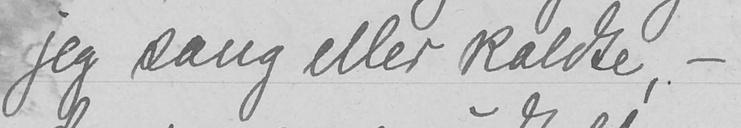jeg sang eller kaldte, -
